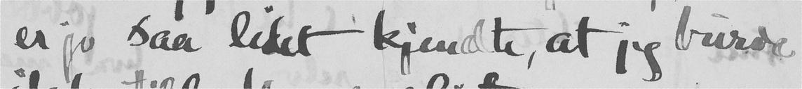er jo saa lidet kjendte, at jeg burde
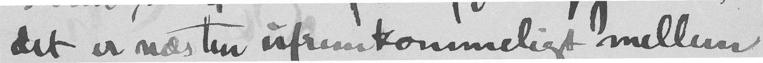det er næsten ufremkommeligt mellem
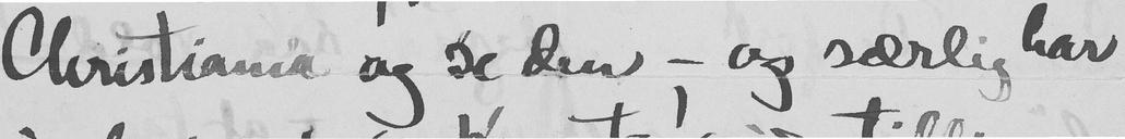Christiania og de den - og særlig har
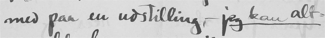med paa en udstilling,- jeg kan alt-
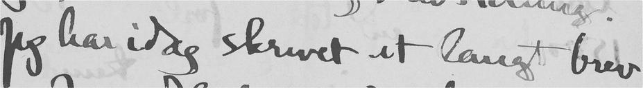Jeg har idag skrevet et langt brev
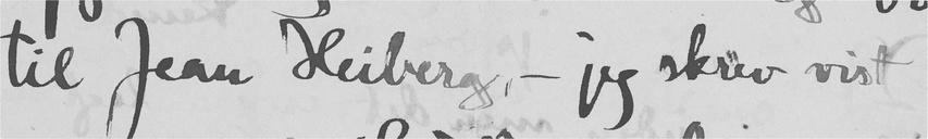til Jean Heiberg, - jeg skrev vist
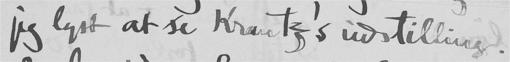jeg lyst at se Krantz's udstilling.
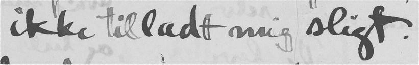ikke tilladt mig sligt.
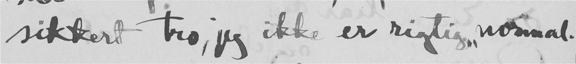sikkert tro, jeg ikke er rigtig "normal.
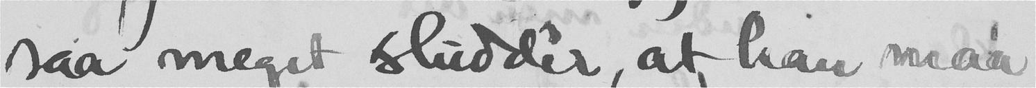saa meget sludder, at han maa
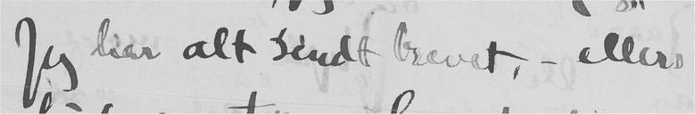Jeg har alt sendt brevet, - ellers
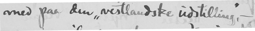med paa den "vestlandske udstilling",-
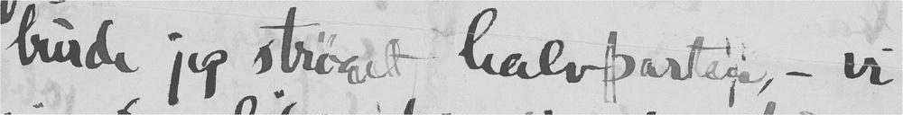burde jeg strøget halvparten, - vi
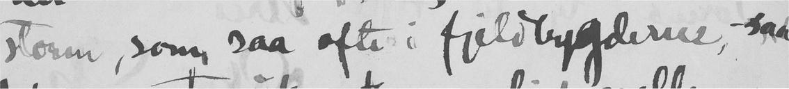storm, som saa ofte i fjeldbygderne, - saa
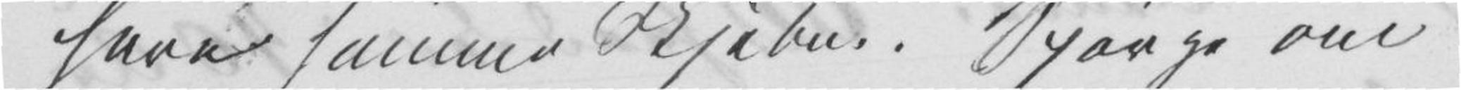have samme Skjebne. Spørge om
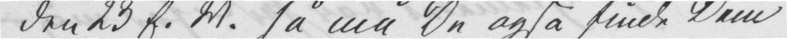den 23 f. M. så må De også finde Dem
